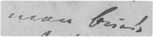man burde
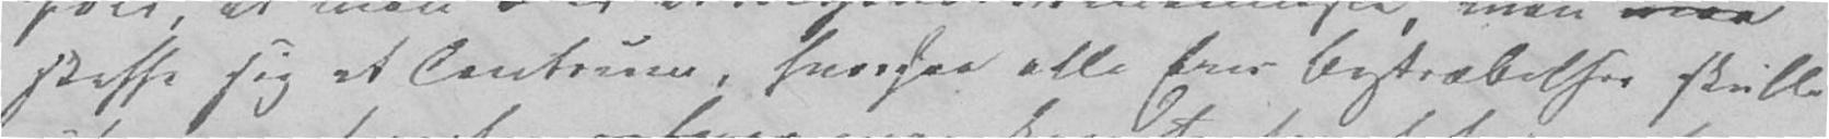skaffe sig et Centrum, hvorfra alle Ens Bestræbelser skulle
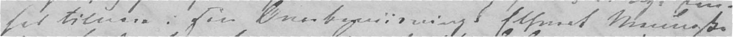hed tilveie i sin Overbeviisning. Ethvert Menneske
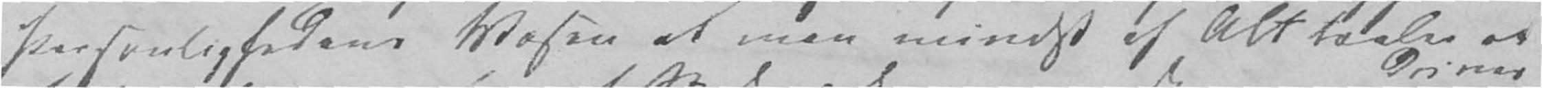Personlighedens Væsen at man mindst af Alt taaler at
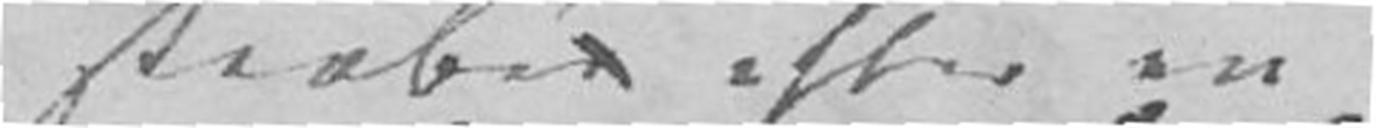stræbe efter en
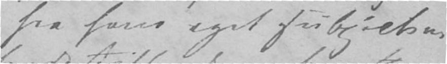fra hans eget subjective
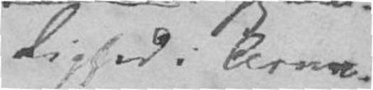Lighed i Arve-
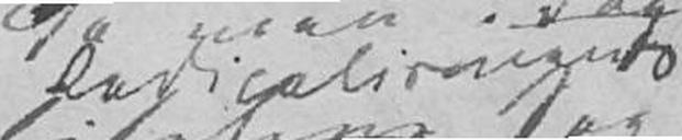Radicalismens
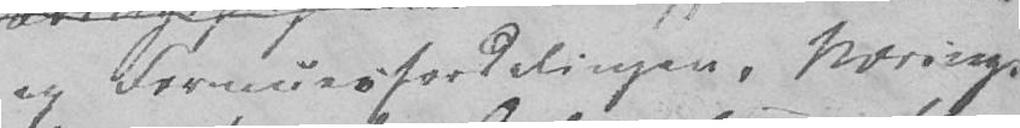og Formuesfordelingen, Nærings-
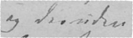og desuden
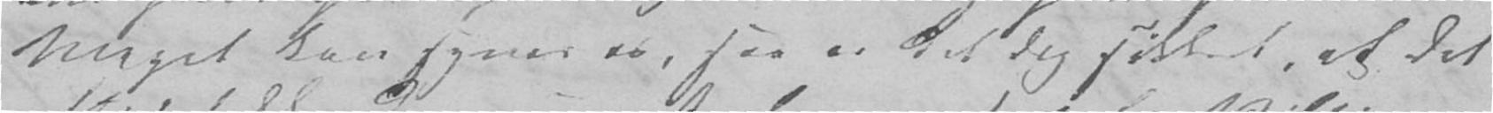Meget kan synes os, saa er det dog sikkert, at det
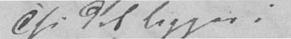Thi det ligger i
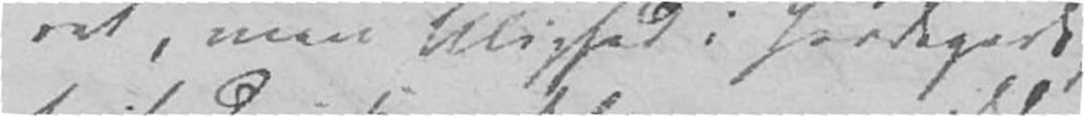ret, men Ulighed i Jordegods
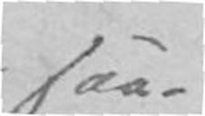saa-
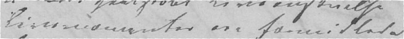Livsmomenter ere formidlede
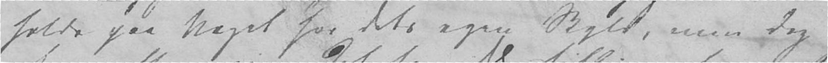holde paa Noget for dets egen Skyld, men dog
